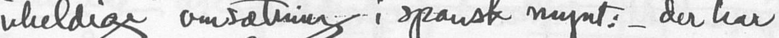uheldige omsætning i spansk mynt: - der har
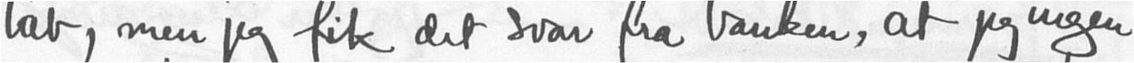tab, men jeg fik det svar fra banken, at jeg ingen
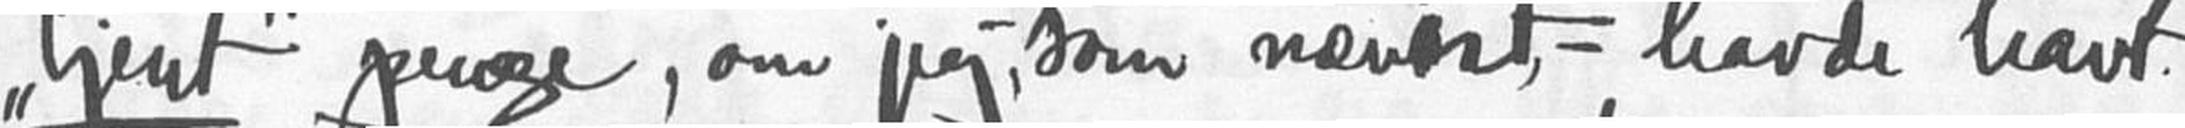"tjent" penge, om jeg som nævnt, havde havt
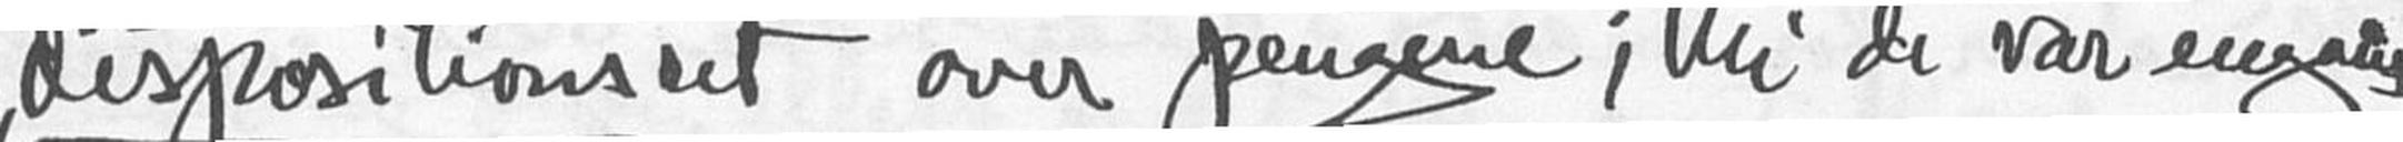"dispositionsret" over pengene, thi de var engang
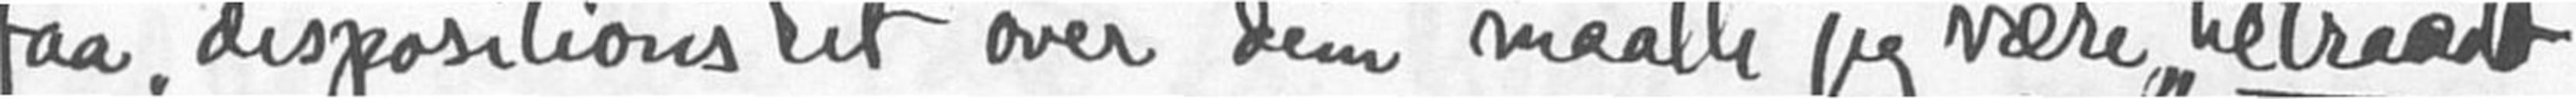faa "dispositionsret" over dem maate jeg være "tiltraadt
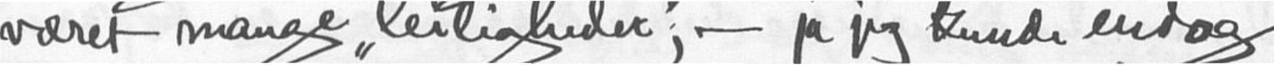været mange "leiligheder"; - ja jeg kunde endog
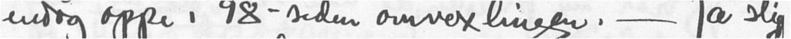endog oppe i 98 - siden omvexlingen. - Ja slig
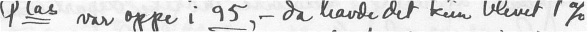Ptas var oppe i 05, - og da havde det kun blevet 1 %
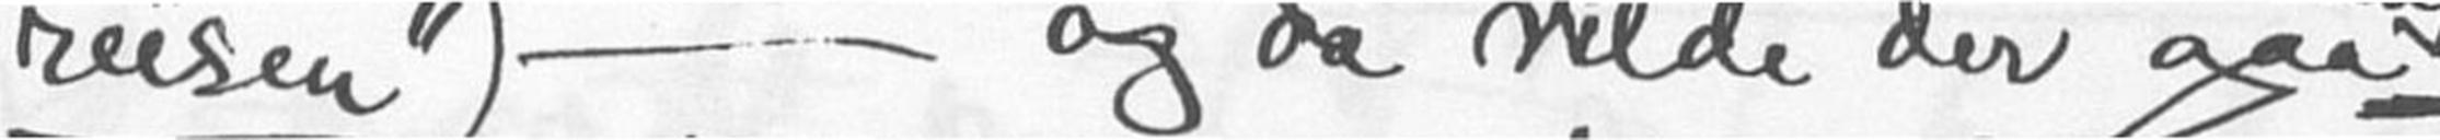reisen") - og da vilde der gaa
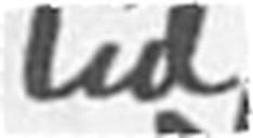tid
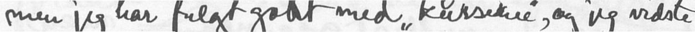men jeg har fulgt godt med "kurserne", og jeg vidste
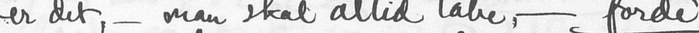er det, - man skal altid tabe, - fordi
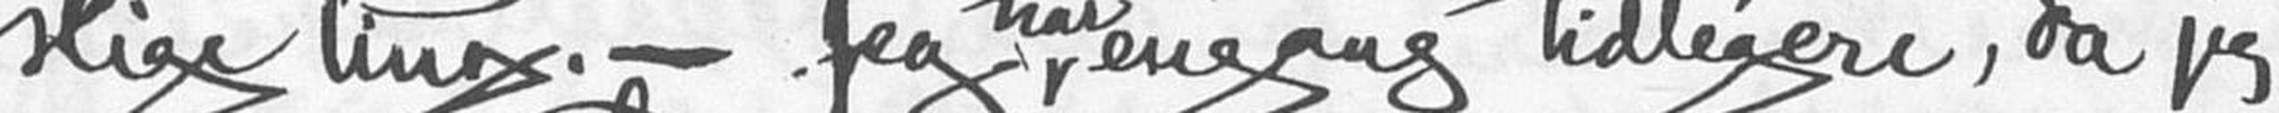slige ting. - Jeg har engang tidligere, da jeg
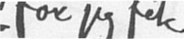før jeg fik
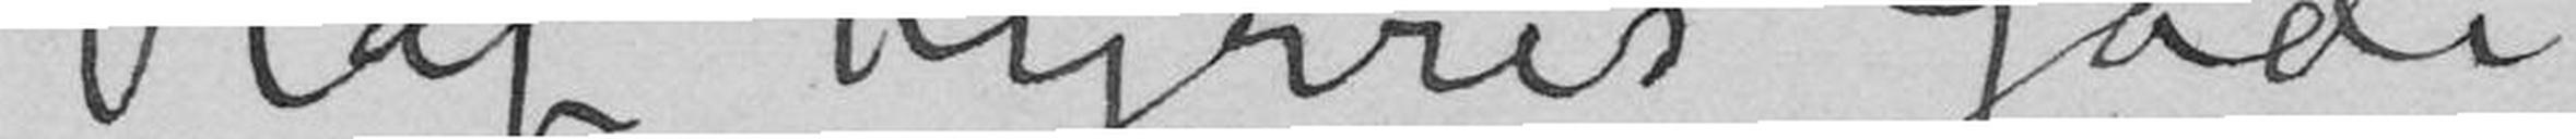Olaf Kyrres Gade
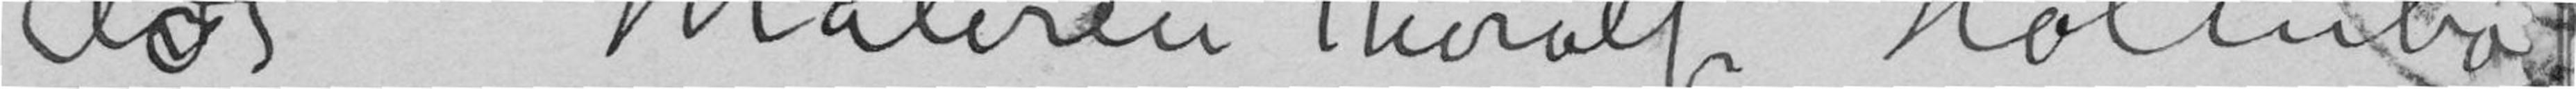Adr Maleren Thorolf Holmboe
